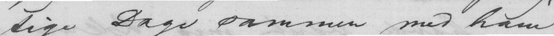tige Dage sammen med ham
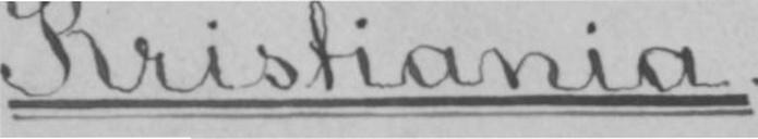Kristiania.
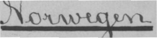Norwegen.
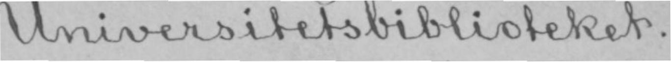Universitetsbiblioteket.
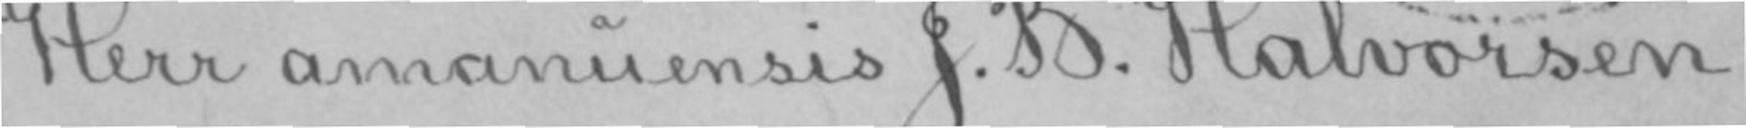Herr amanuensis J. B. Halvorsen.
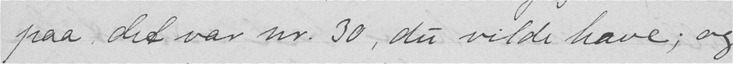paa, det var nr. 30, du vilde have; og
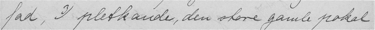fad, 3) pletkande, den store gamle pokal
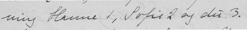ning Hanne 1, Sofie 2 og du 3.
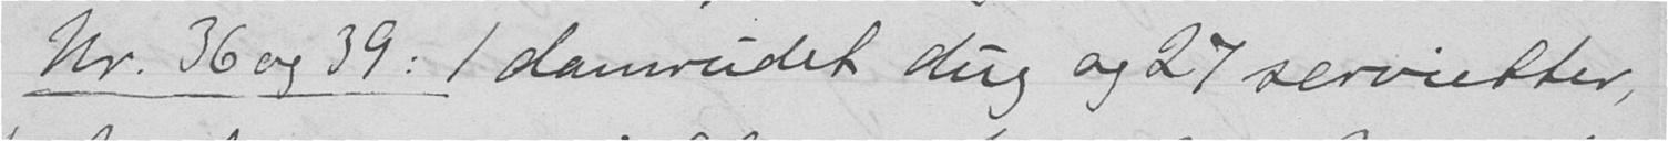Nr. 36 og 39: 1 damrudet dug og 27 servietter,
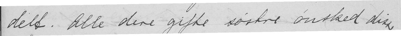delt. Alle dere gifte søstre ønsked disse
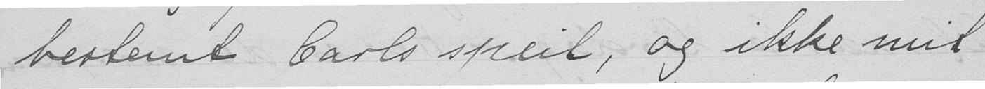bestemt Carls speil, og ikke mit
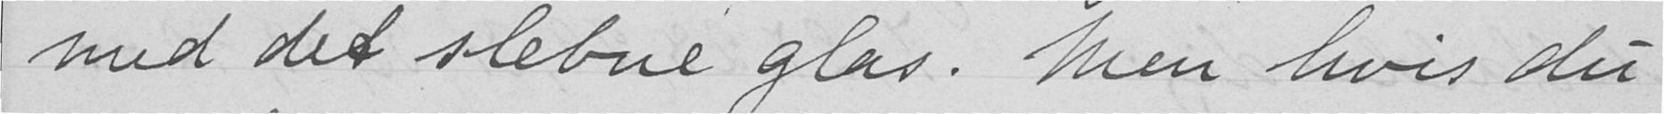med det slebne glas. Men hvis du
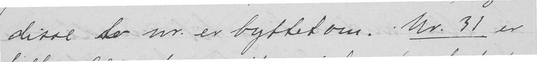disse to nr. er byttet om. Nr. 31 er
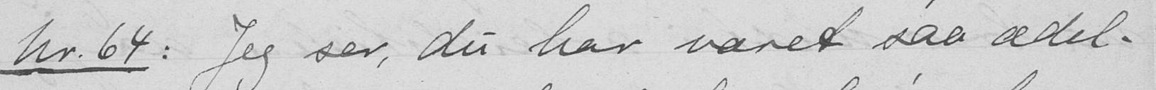Nr. 64: Jeg ser, du har været saa ædel-
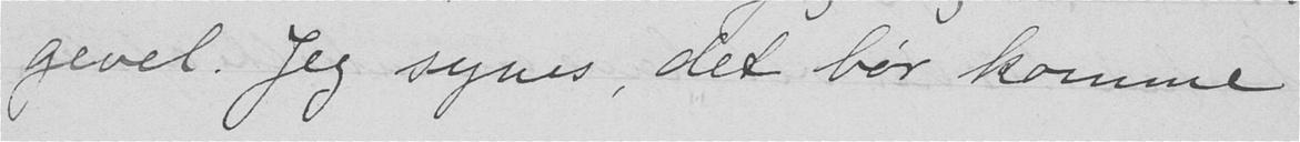gevel. Jeg synes, det bør komme
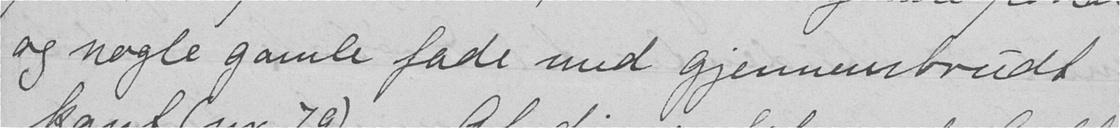og nogle gamle fade med gjennembrudt
